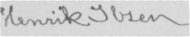Henrik Ibsen.
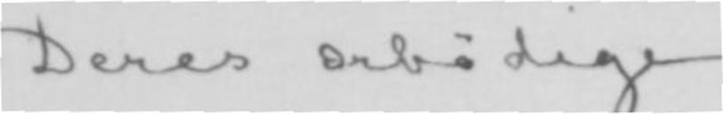Deres ærbødige
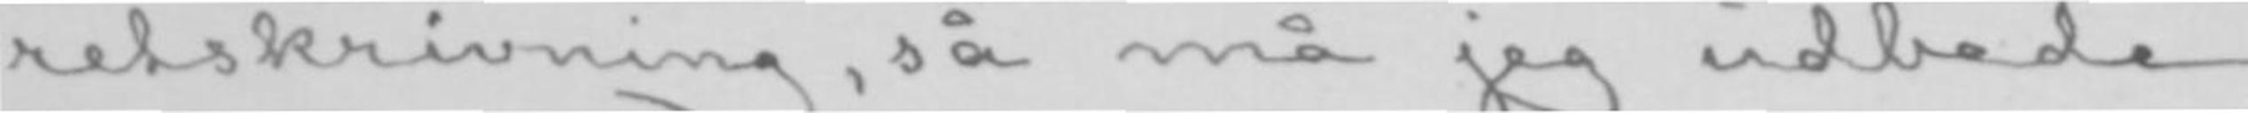retskrivning, så må jeg udbede
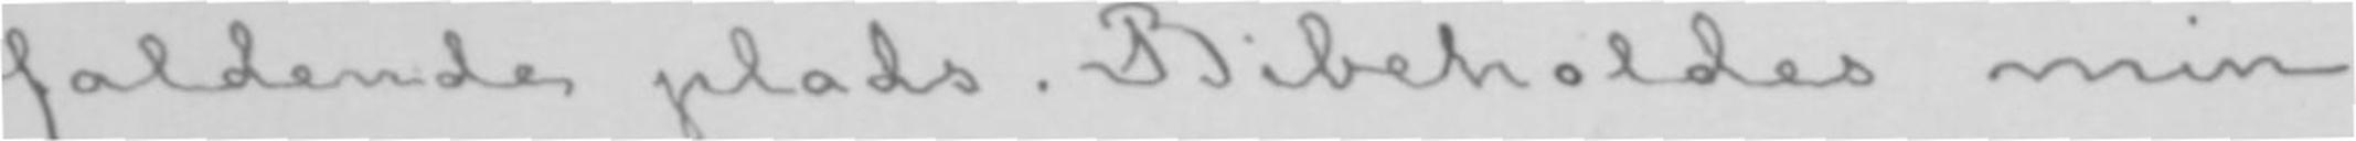faldende plads. Bibeholdes min
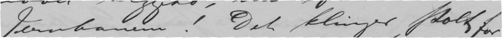Jernbanen! Det klinger stolt for
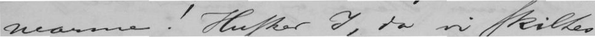nearme! Husker I, da vi skiltes
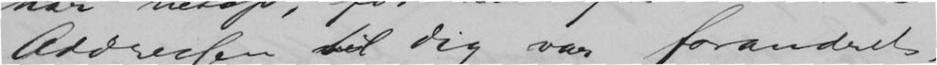Addressen til dig var forandret,
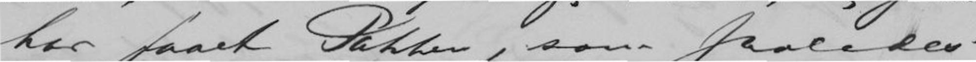har faaet Pakken, som saaledes
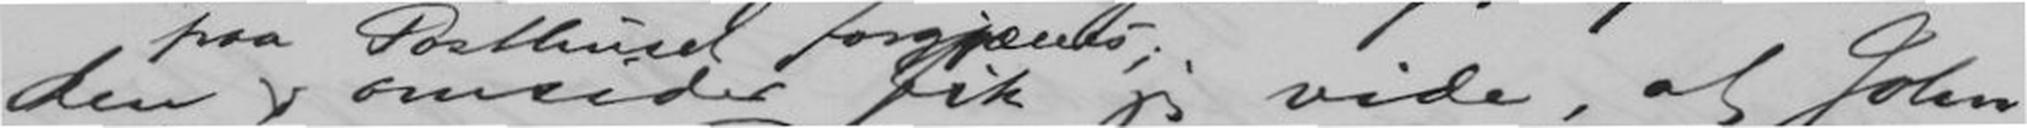den omsider fik jeg vide, at John
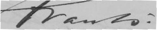Frants.
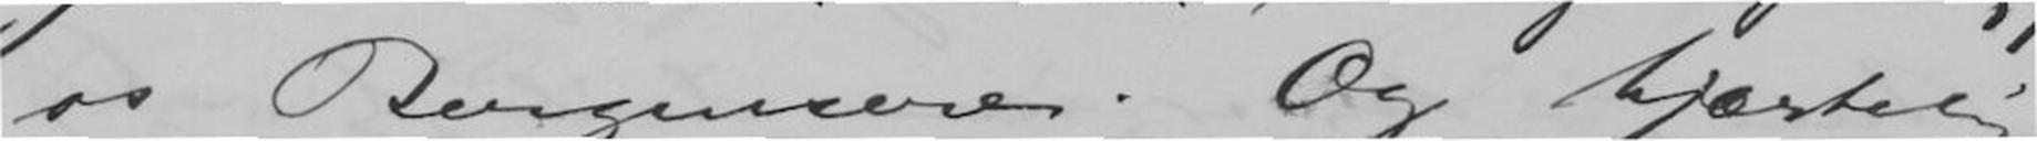os Bergensere. Og hjærtelig
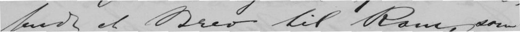sendt et Brev til Rom, som
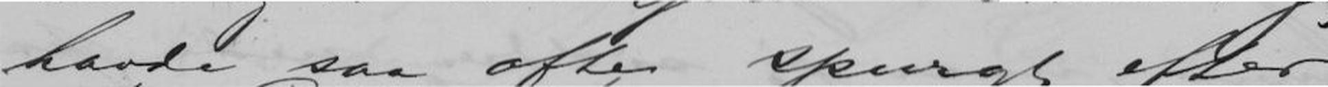havde saa ofte spurgt efter
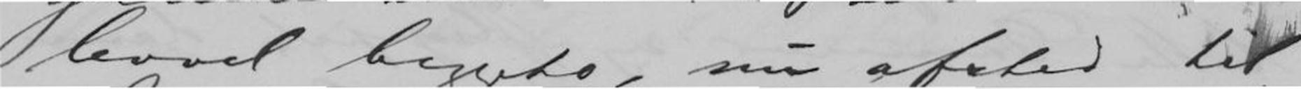levvel beggeto, nu afsted til
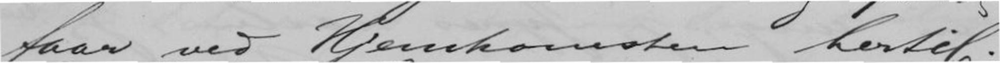faar ved Hjemkomsten hertil.
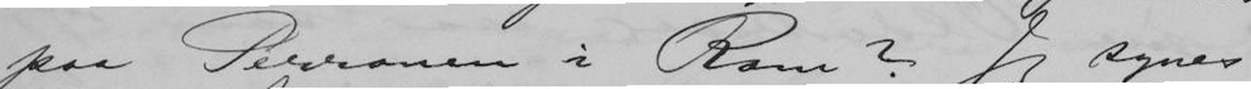paa Perronen i Rom? Jeg synes
